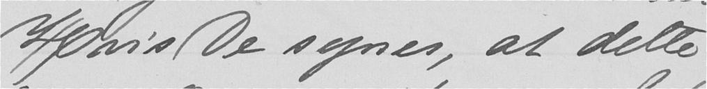Hvis De synes, at dette
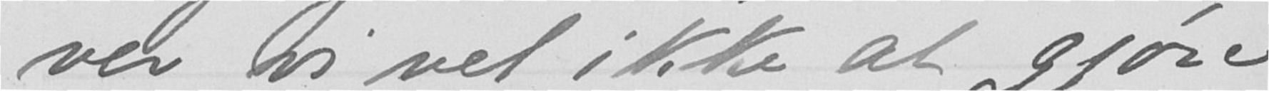ver vi vel ikke at gjøre
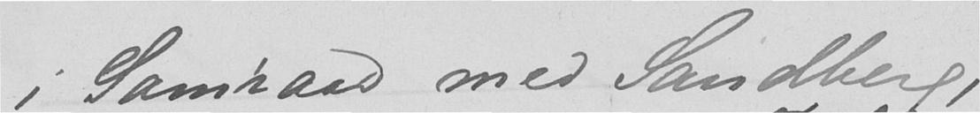i Samraad med Sandberg,
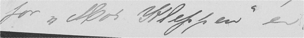for "Mor Kleppen" er
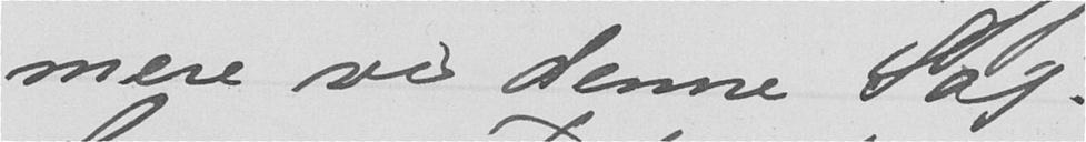mere ved denne Sag.
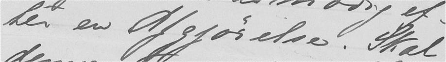ter en Afgjørelse. Skal
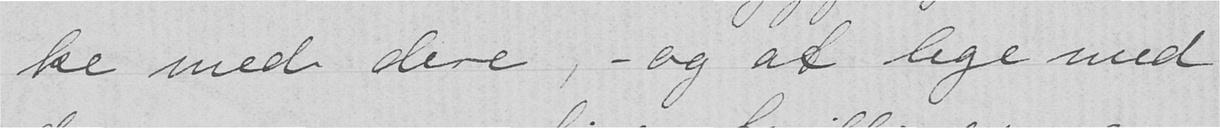ke med dere, - og at lege med
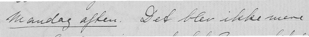Mandag aften. Det blev ikke mere
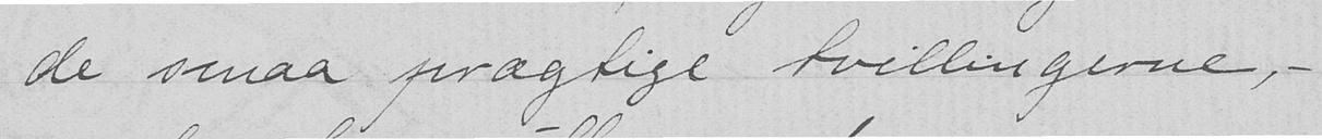de smaa prægtige tvillingerne, -
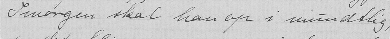Imorgen skal han op i mundtlig,
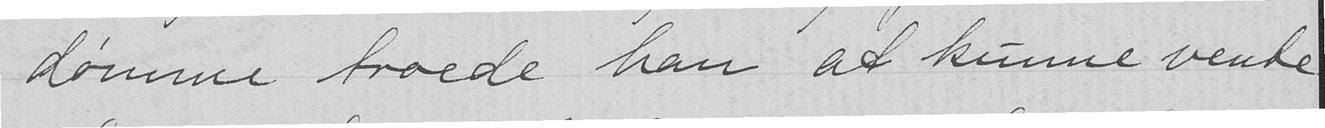dømme troede han at kunne vente
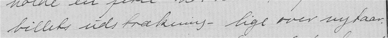billets udstrækning - lige over nytaar.
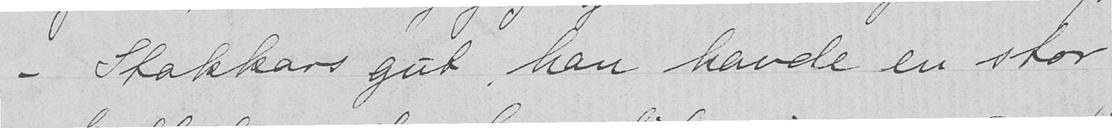- Stakkars gut, han havde en stor
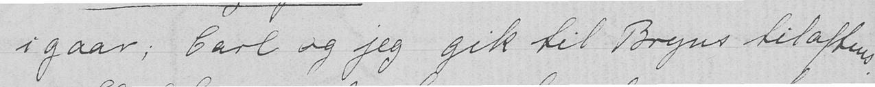igaar; Carl og jeg gik til Bryns tilaftens.
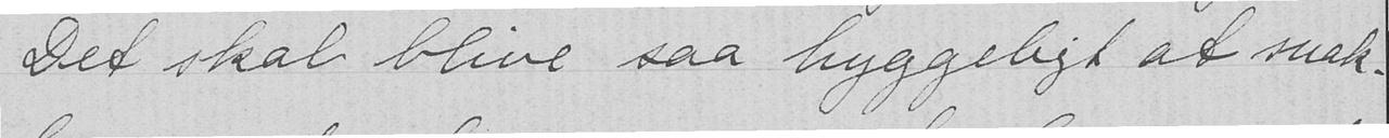Det skal blive saa hyggeligt at snak-
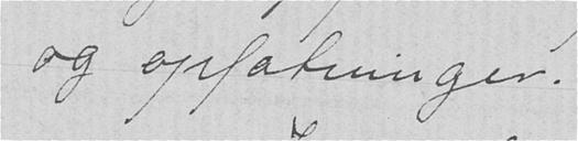og opfatninger.
